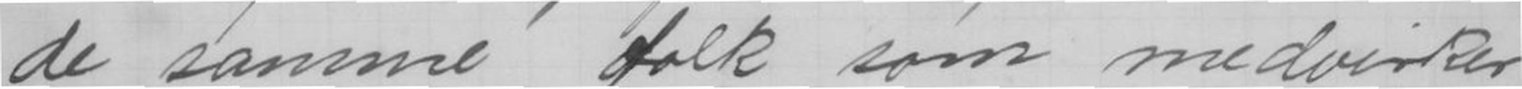de samme folk som medvirker
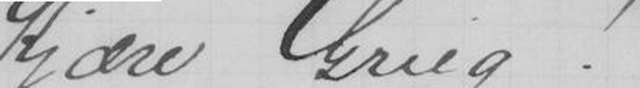Kjære Grieg!
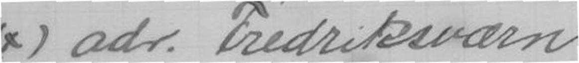(x) adr. Fredriksværn
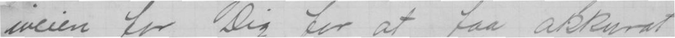iveien for Dig for at faa akkurat
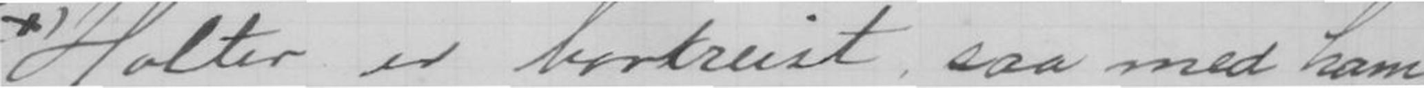Holter er bortreist, saa med ham
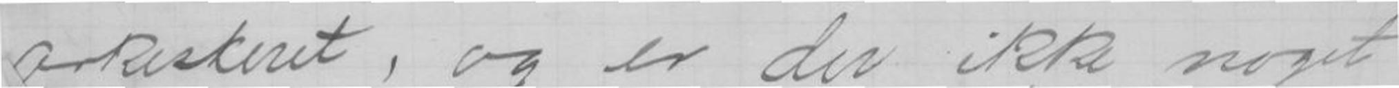orkesteret, og er der ikke noget
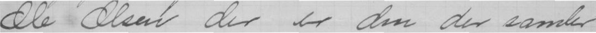Ole Olsen der er den der samler
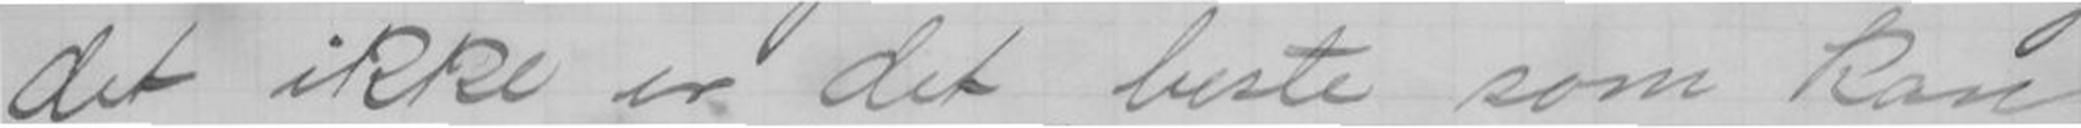det ikke er det bedste som kan
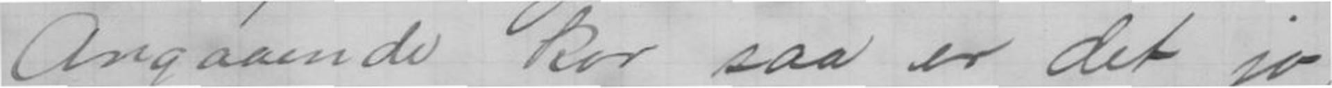Angaaende kor saa er det jo,
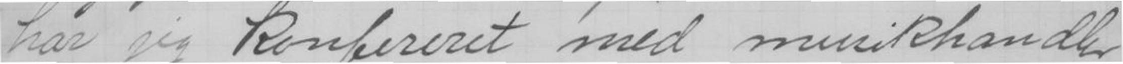har jeg konfereret med musikhandler
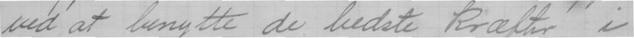ved at benytte de bedste kræfter i
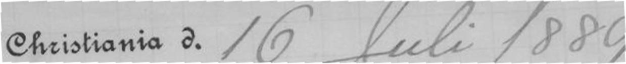Christiania d. 16 Juli 1889.
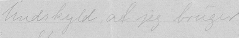Undskyld: at jeg bruger
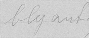blyant.
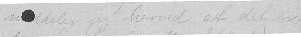meddeler jeg. herved, at det er,
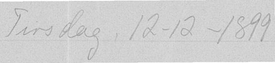Tirsdag , 12-12-1899
